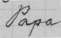Papa
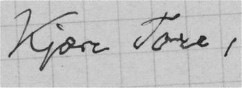Kjære Tore,
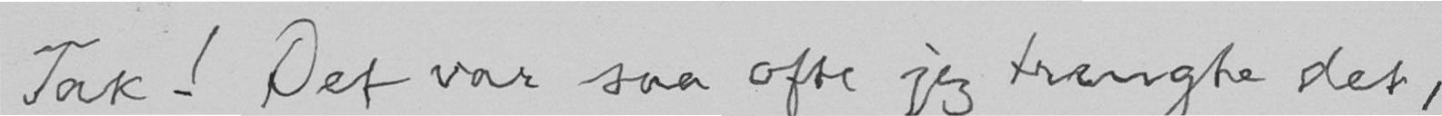Tak! Det var saa ofte jeg trengte det,
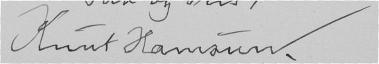Knut Hamsun.
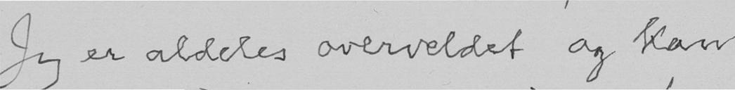Jeg er aldeles overveldet og kan
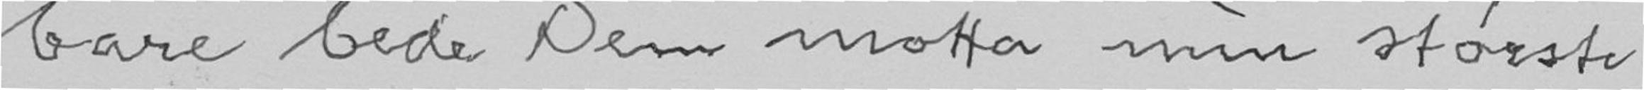bare bede Dem motta min største
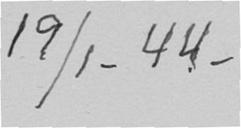19/1. 44.
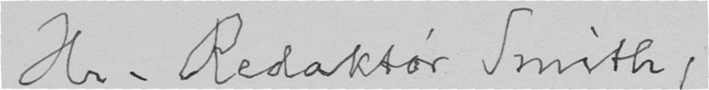Hr. Redaktør Smith,
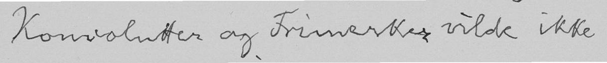Konvolutter og Frimerker vilde ikke
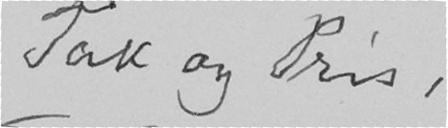Tak og Pris,
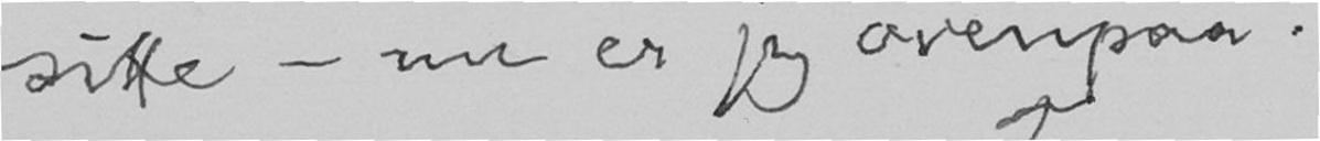sitte - nu er jeg ovenpaa.
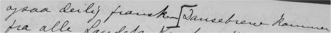ogsaa deilig fransk. Dansebreve kommer
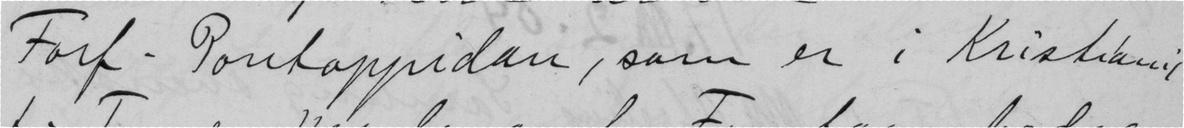Forf. Pontoppidan, som er i Kristiania
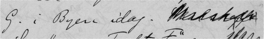G. i Byen idag.
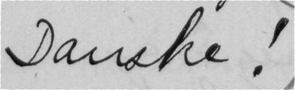Danske!
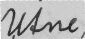Utne
